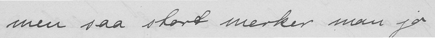men saa stort merker man jo
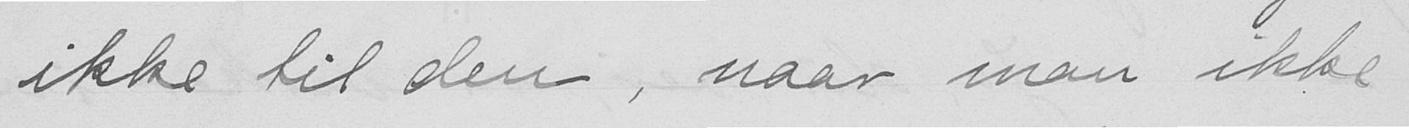ikke til den, naar man ikke
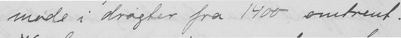møde i dragter fra 1400 omtrent.
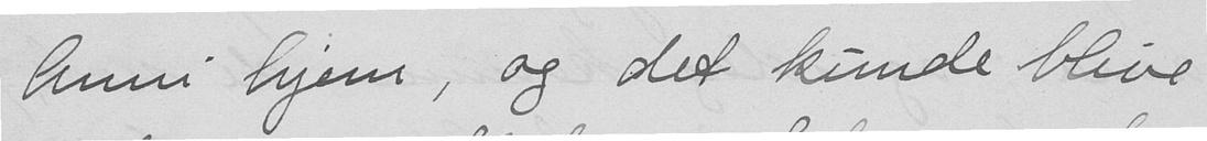Anni hjem, og det kunde blive
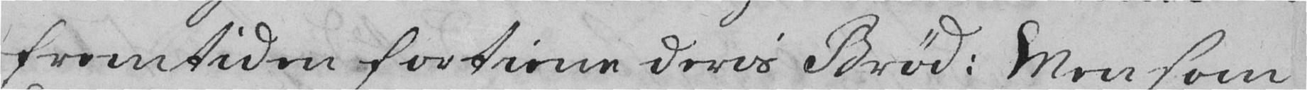fremtiden fortiene deris Brød: Men som
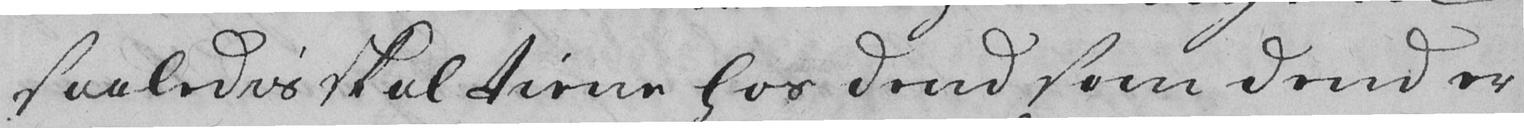saaledis skal tiene hos dend som dend er
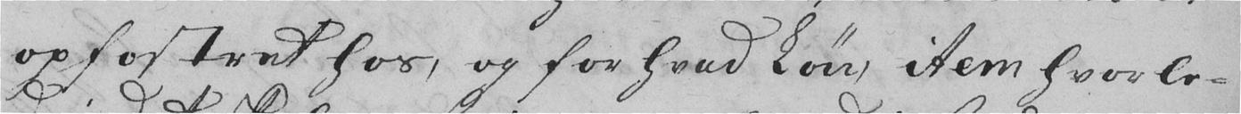opfostret hos, og for hvad Løn, item hvorle-
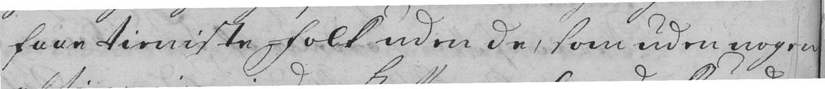faae tieneste-Folk uden de, som uden nogen
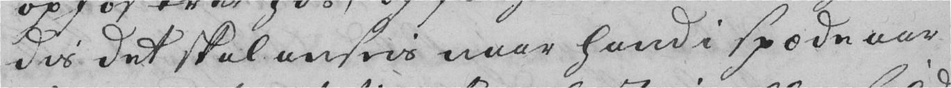dis det skal anseis naar hand i spæde aar
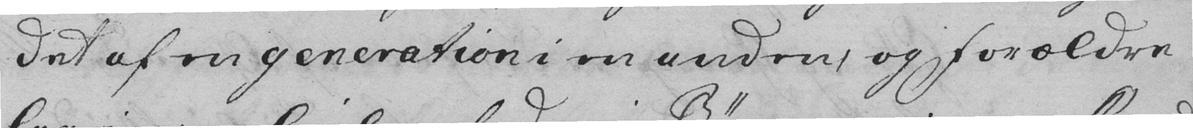det af en generation i en anden, og Forældre
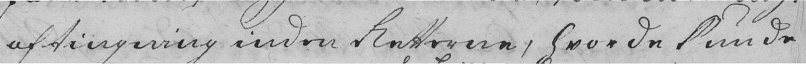aftingning inden Retterne, hvor de kunde
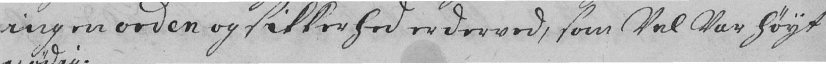ingen orden og sikkerhed er derved, som Vel Var høyt
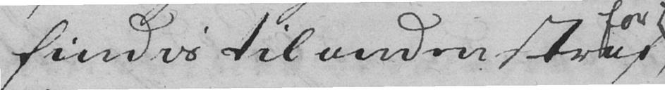findis til anden straf
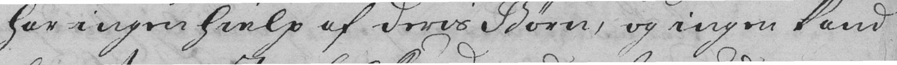har ingen hielp af deris Børn, og ingen kand
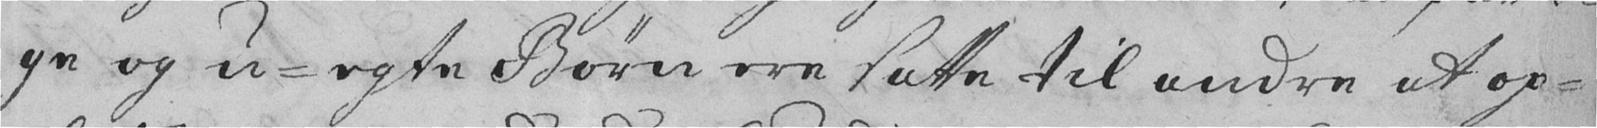ge og u-egte Børn ere satte til andre at op-
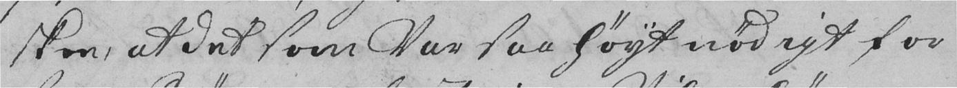skee, at det som var saa høyt nødigt for
